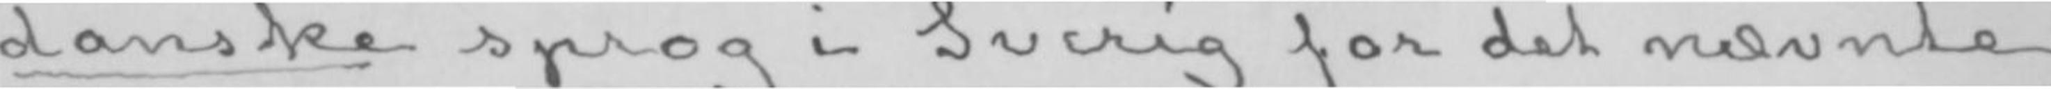danske sprog i Sverig for det nævnte
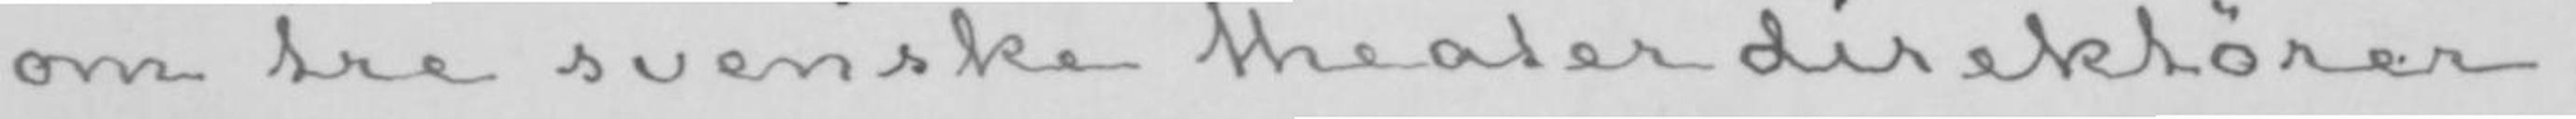om tre svenske theaterdirektører,
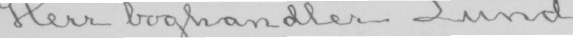Herr boghandler Lund.
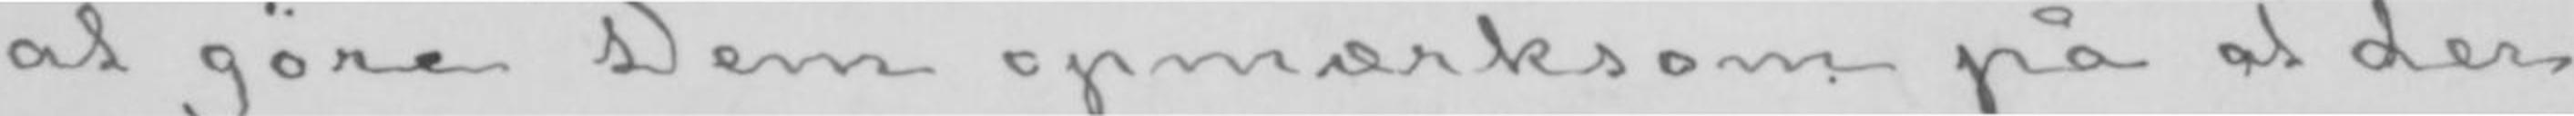at gøre Dem opmærksom på at der
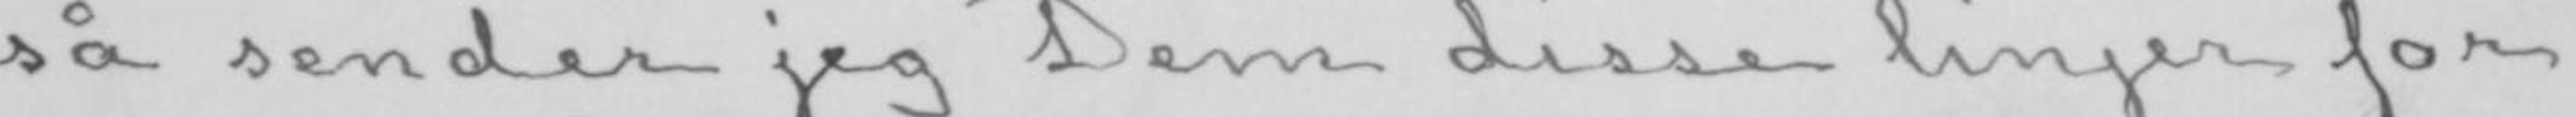så sender jeg Dem disse linjer for
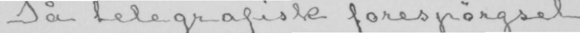På telegrafisk forespørgsel
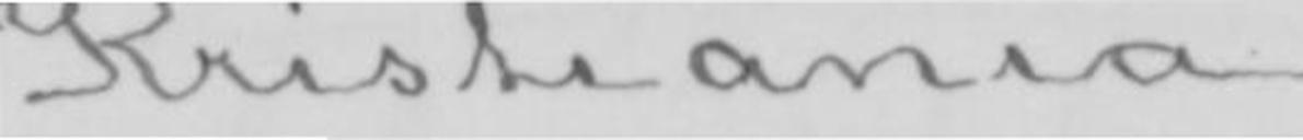Kristiania.
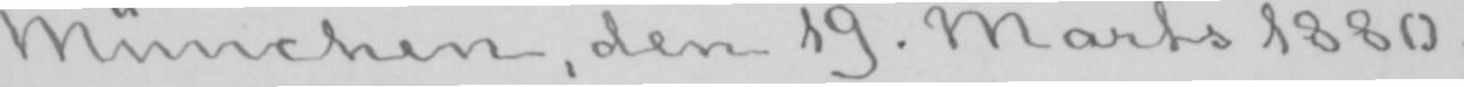München, den 19. Marts 1880.
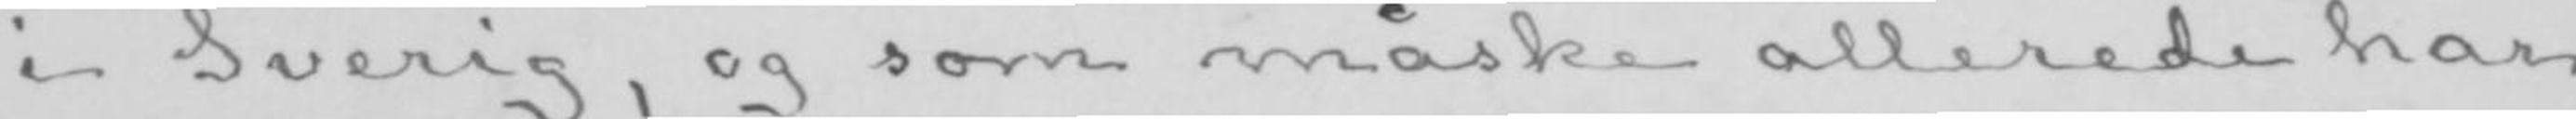i Sverig, og som måske allerede har
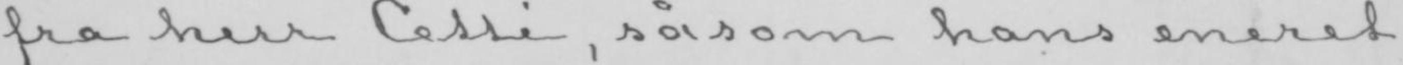fra herr Cetti, såsom hans eneret
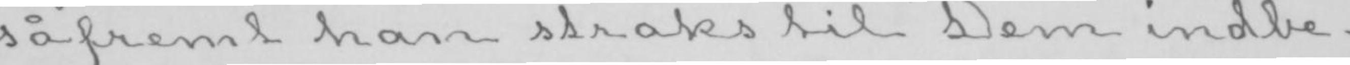såfremt han straks til Dem indbe-
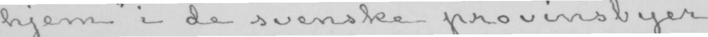hjem» i de svenske provinsbyer
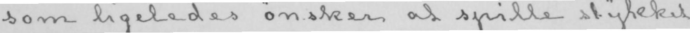som ligeledes ønsker at spille stykket
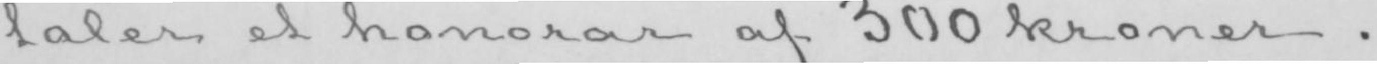taler et honorar af 300 kroner.
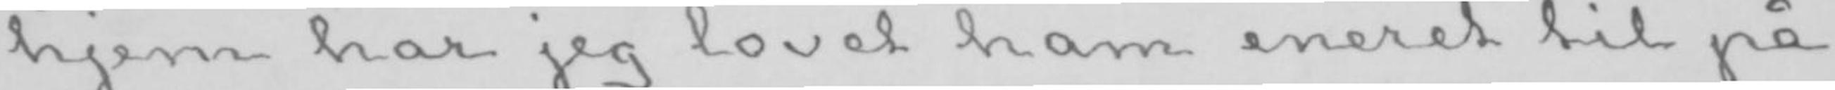hjem har jeg lovet ham eneret til på
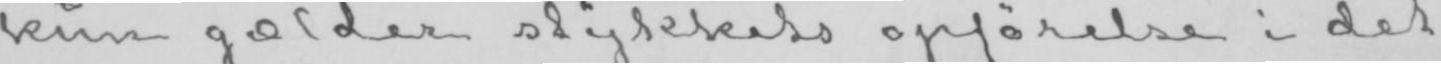kun gælder stykkets opførelse i det
